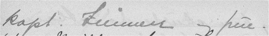kapt. Zimmer og frue.
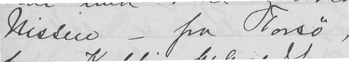Nissen - fra Torsø,
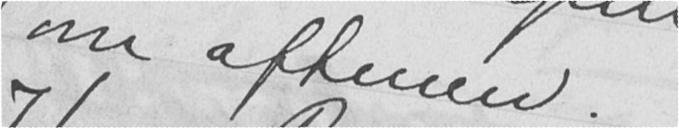om aftenen.
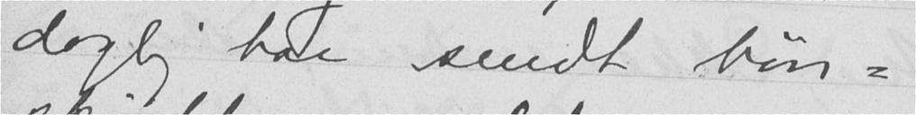daglig har sendt bøn-
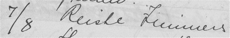7/8 Reiste Zimmers
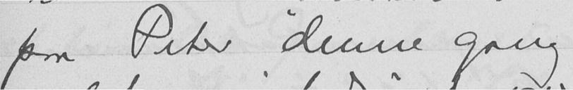paa Peter denne gang
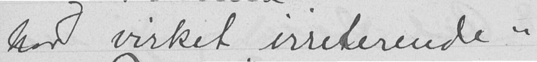har virket "irriterende"
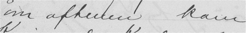om aftenen kom
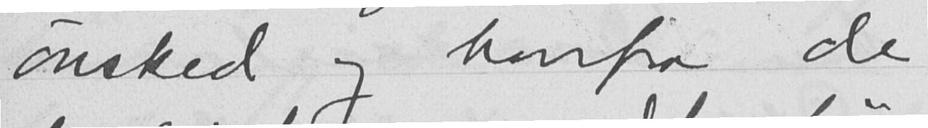ønsked og hvorfra de
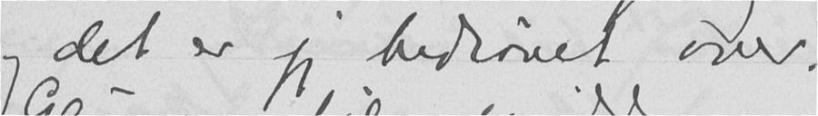og det er jeg bedrøvet over.
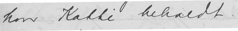hvor Katti beholdt
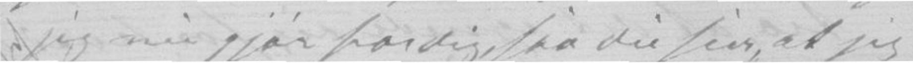jeg nu gjør færdig, saa du ser, at jeg
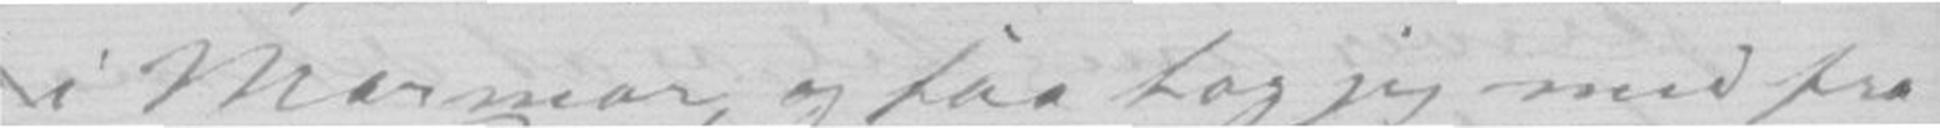i Marmor, og saa tog jeg med fra
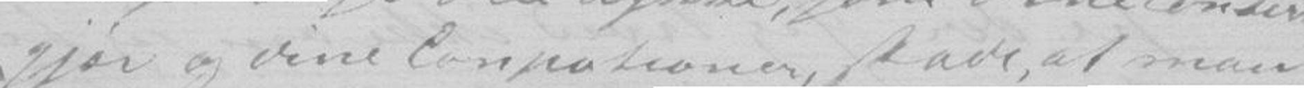gjør og dine Compotioner, skade, at man
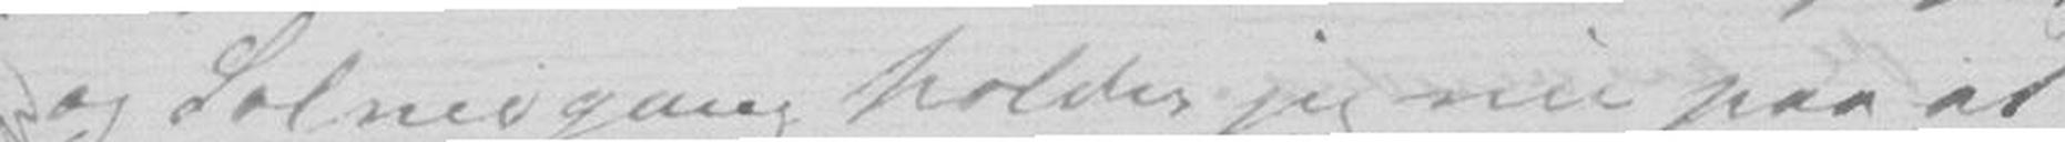og Solnedgang holder jeg nu paa at
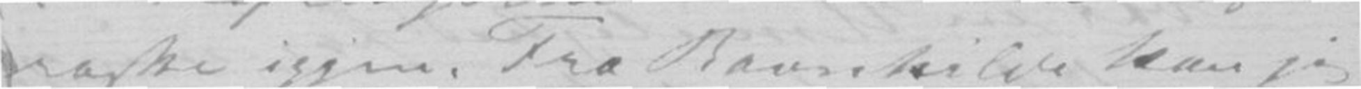raske igjen. Fra Ravnkilde kan jeg
